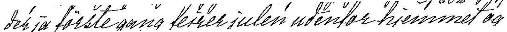der jo første gang feirer julen udenfor hjemmet og
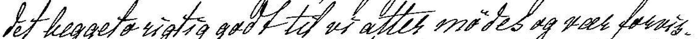det beggeto rigtig godt til vi atter mødes og vær forvis-
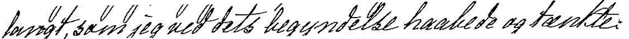langt, som jeg ved dets begyndelse haabede og tænkte.
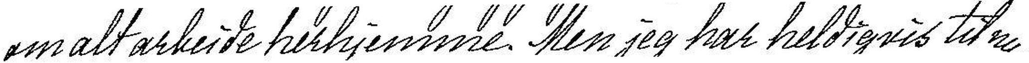om alt arbeide herhjemme. Men jeg har heldigvis til nu
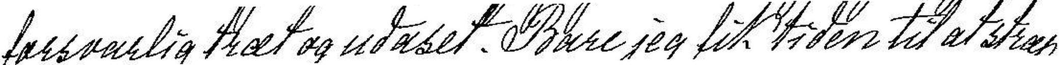forsvarlig træt og udaset. Bare jeg fik tiden til at stræk-
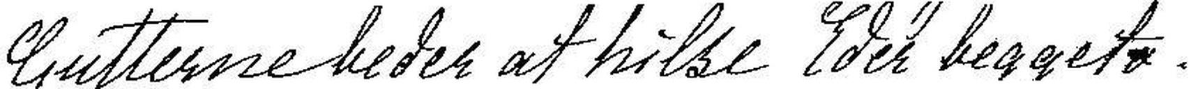Gutterne beder at hilse begge to.
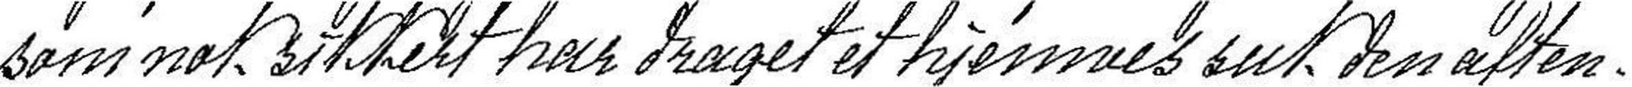som nok sikkert har draget et hjemmes suk den aften.
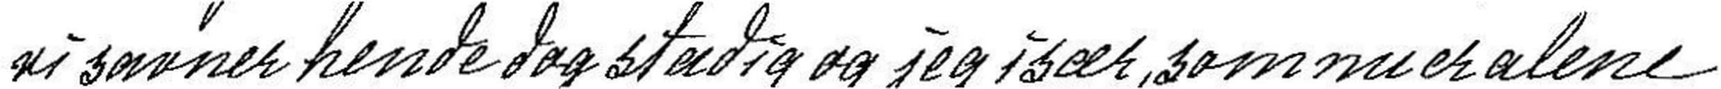vi savner hende dog stadig og jeg især, som nu er alene
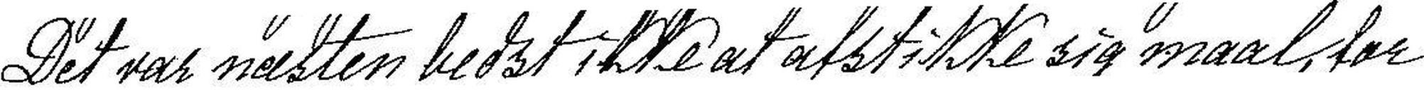Det var næsten bedst ikke at afstikke sig maal, for
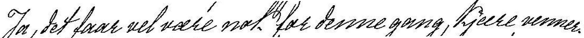Ja, det faar vel være nok for denne gang, kjære venner.
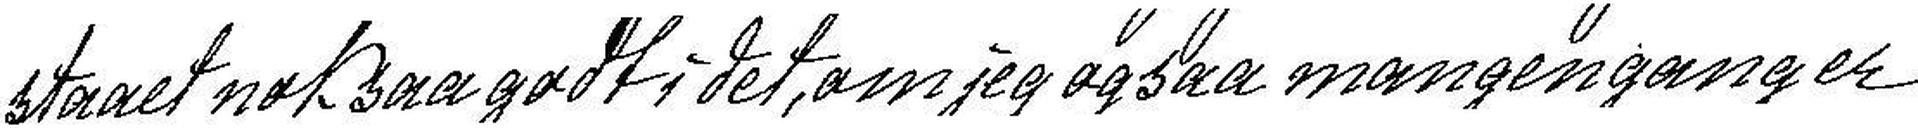staaet noksaa godt i det, om jeg ogsaa mangen gang er
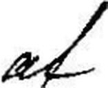af
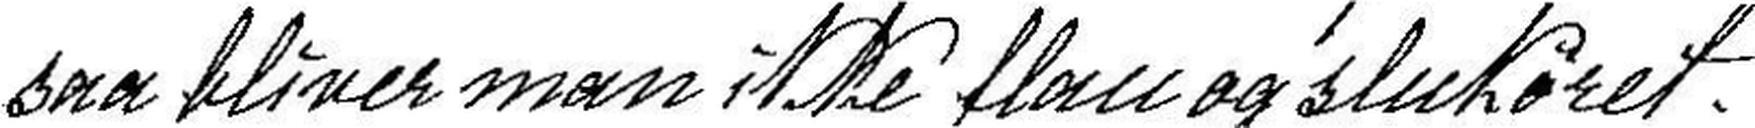saa bliver man ikke flau og slukøret.
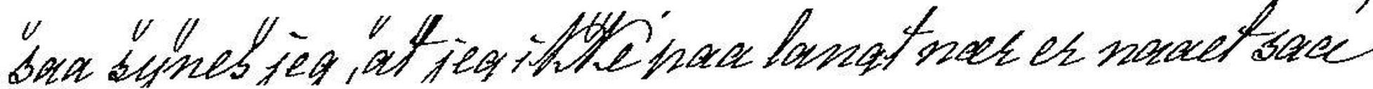saa synes jeg, at jeg ikke paa langt nær er naaet saa
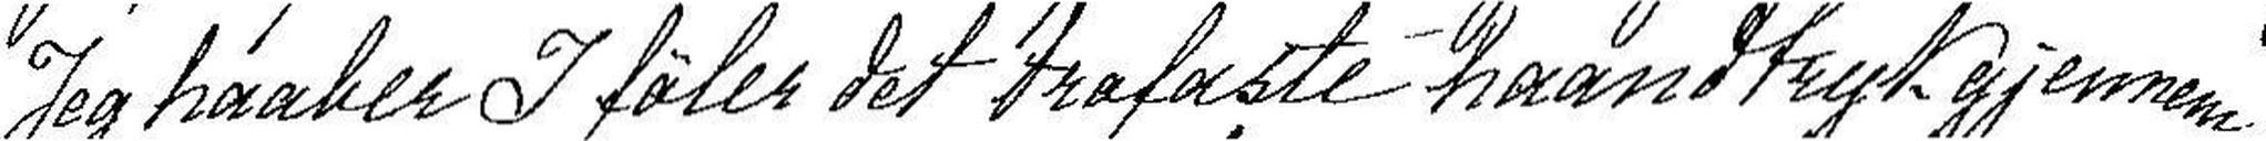Jeg haaber I føler det trofaste haandtryk gjennem
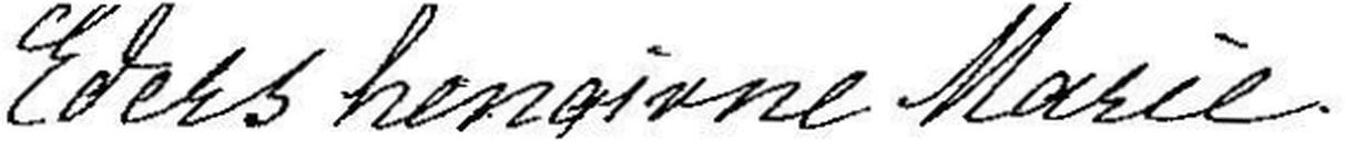Eders hengivne Marie
